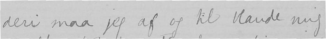deri maa jeg af og til blande mig
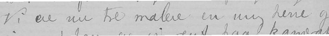Vi ere nu tre malere en ung herre og
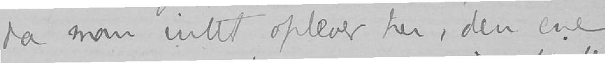da man intet oplever her, den ene
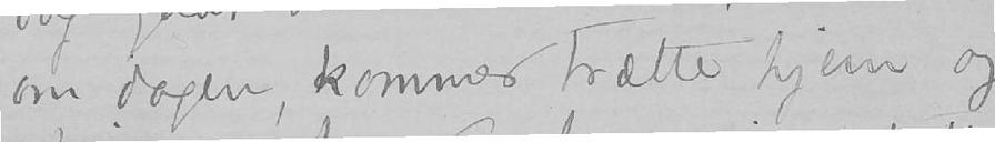om dagen, kommer trætte hjem og
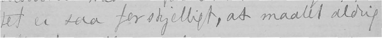tet er saa forskjelligt, at maalet aldrig
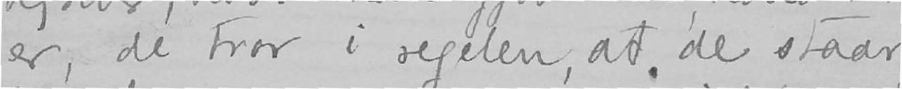er, de tror i regelen, at de staar
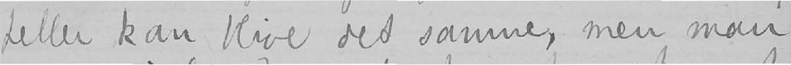heller kan blive det samme, men man
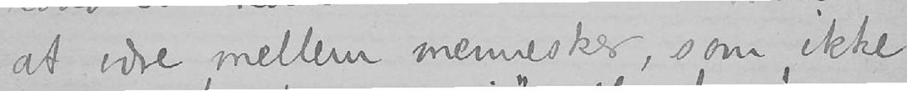at være mellem mennesker, som ikke
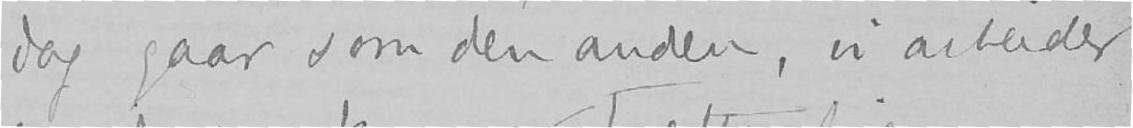dag gaar som den anden, vi arbeider
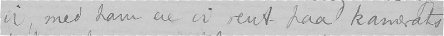vi, med ham ere vi rent paa kamerats-
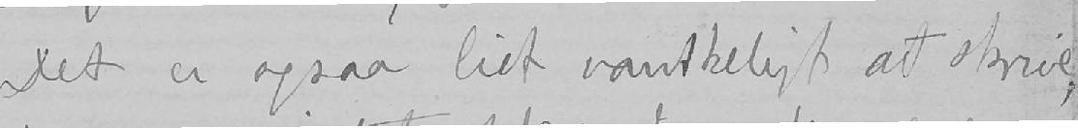Det er ogsaa lidt vanskeligt at skrive,
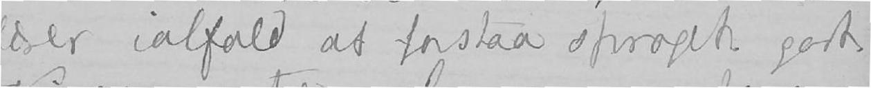lærer ialfald at forstaa sproget godt.
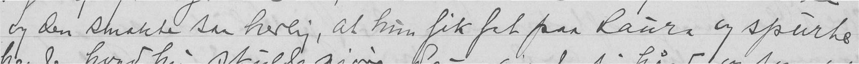og den smakte saa herlig, at hun fik fat paa Laura og spurte
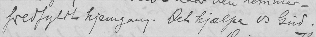fredfyldt hjemgang. Det hjælpe os Gud.
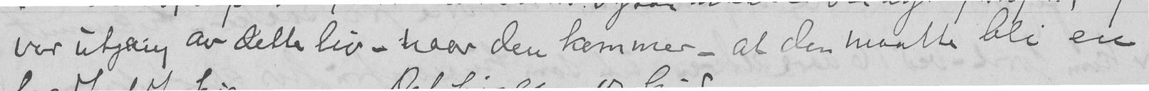vor utgang av dette liv - naar den kommer - at den maatte bli en
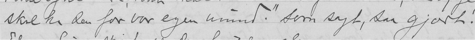skal ha den for vor egen mund." Som sagt, saa gjort.
